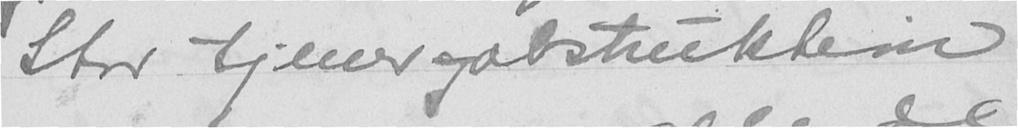Stor tjener-obstruktion
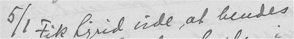5/1 Fik Sigrid vide, at hendes
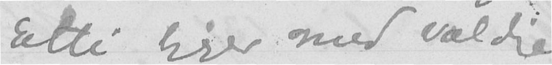Etti ligger med veldige
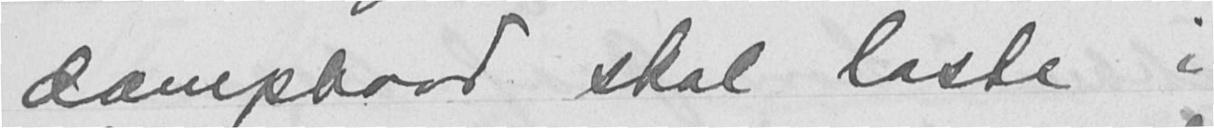dampbaad skal laste i
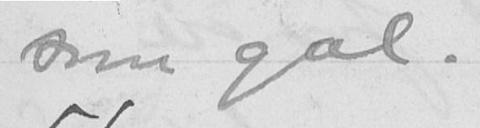som gal.
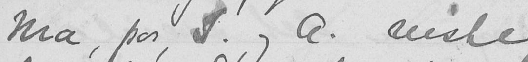Ma, pa, J. og A. reiste
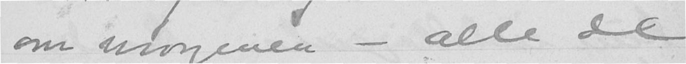om morgenen - alle de
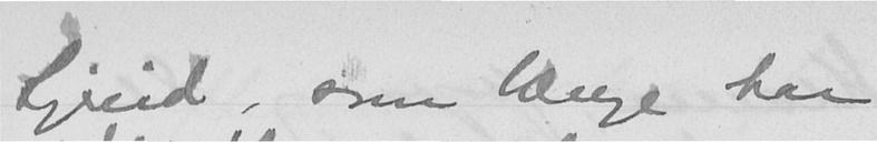Sigrid, som længe har
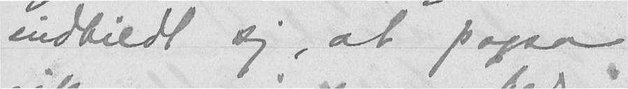indbildt sig, at papa
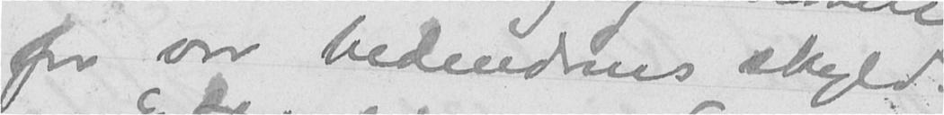for vor hedendoms skyld.
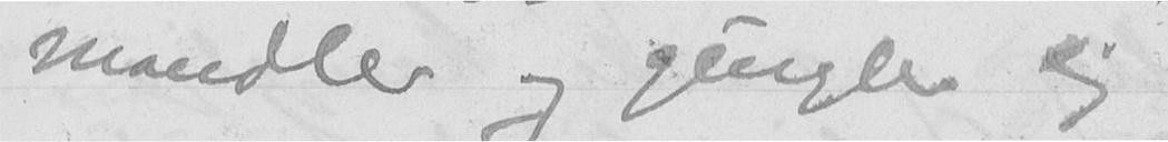mandler og gurgler sig
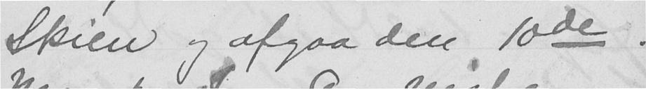Skien og afgaa den 10de.
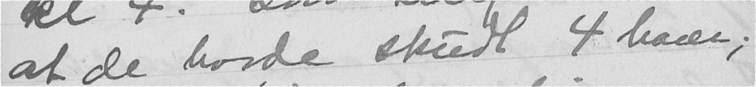at de havde skudt 4 harer,
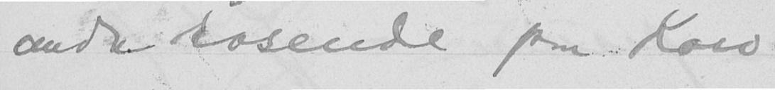andre rasende paa Koss
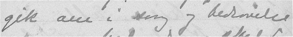gik om i sorg og bedrøvelse
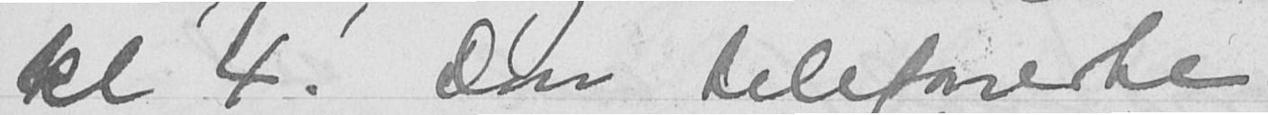kl. 4. Don telefonerte
